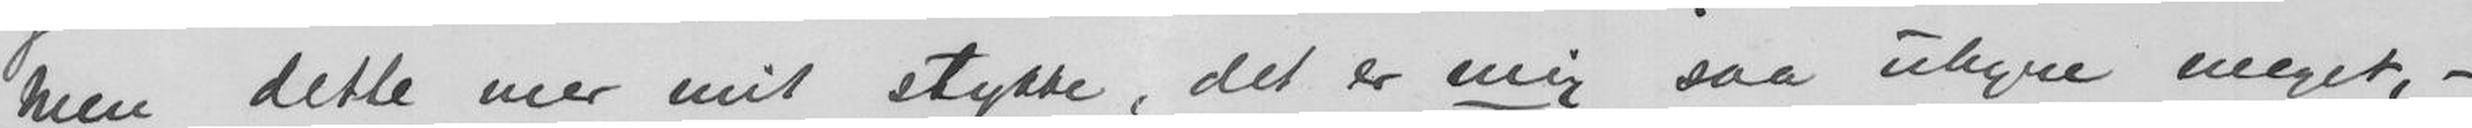Men dette mer mit stykke, det er mig saa uhyre meget, -
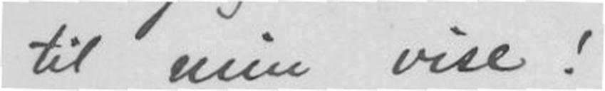til min vise!
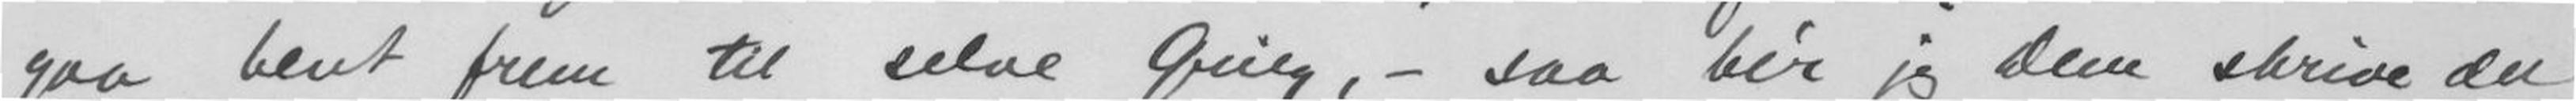gaa bent frem ti selve Grieg, - saa ber jeg Dem skrive det
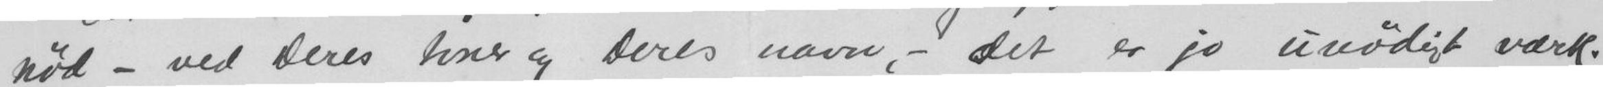nød - ved Deres toner og Deres navn, - det er jo unødigt værk.
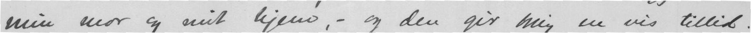min mor og mit hjem, - og den gir mig en vis tillit.
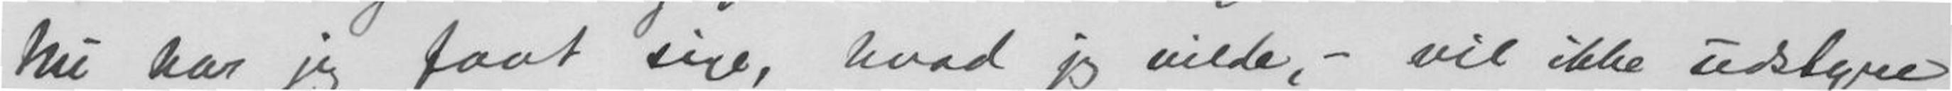Nu har jeg faat sige, hvad jeg vilde, - vil ikke udstyre
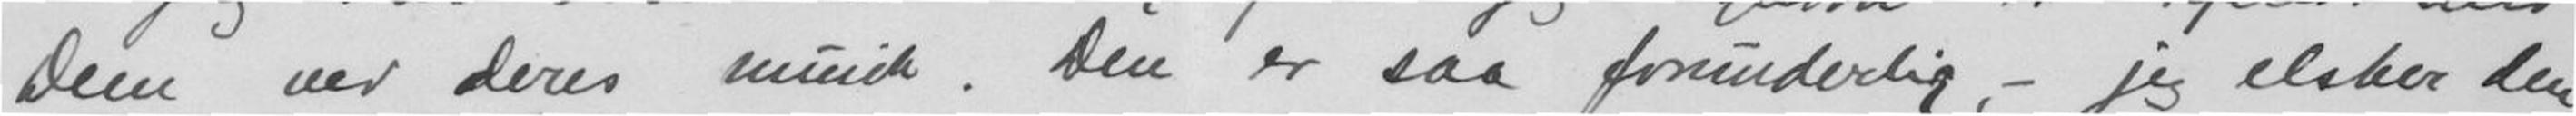Dem ved Deres musik. Den er saa forunderlig, - jeg elsker den
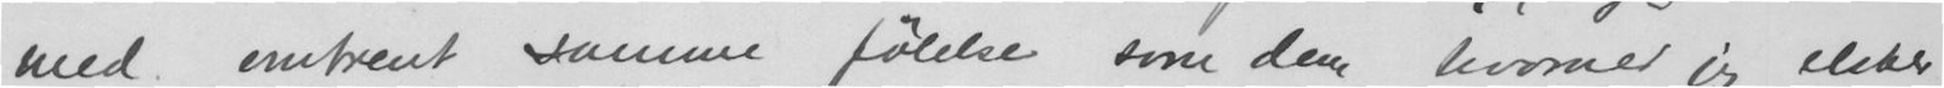med omtrent samme følelse som den kvinne jeg elsker
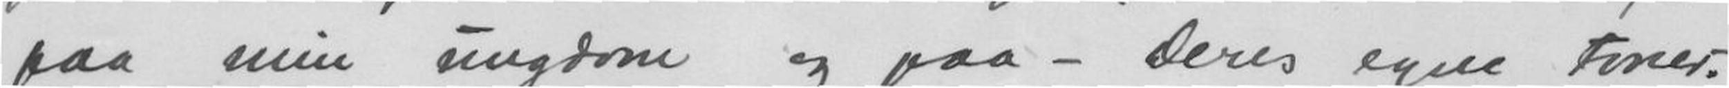paa min ungdom og paa - Deres egne Toner.
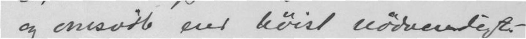og omsvøb end høist nødvendigt -
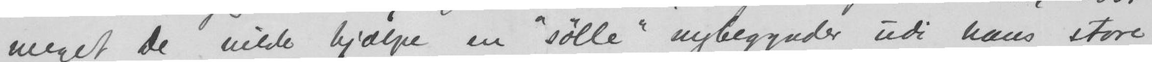meget De vilde hjælpe en "sølle" nybegynder udi hans store
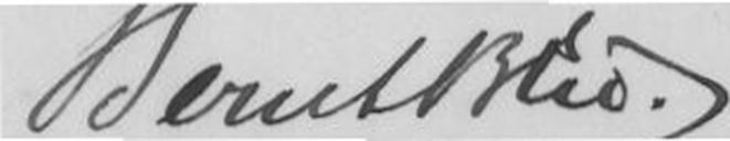Bernt B. Lie.
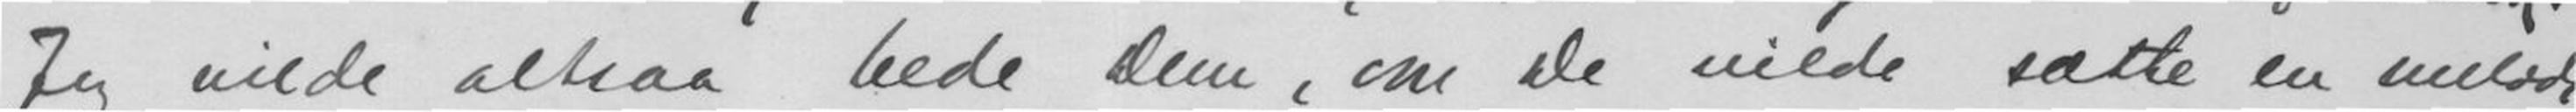Jeg vilde altsaa bede Dem, om De vilde sætte en melodi
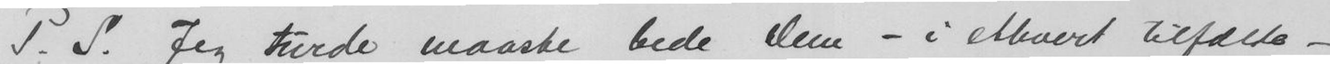P. S. Jeg turde maaske bede Dem - i ethvert tilfælde -
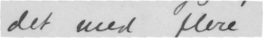det med flere
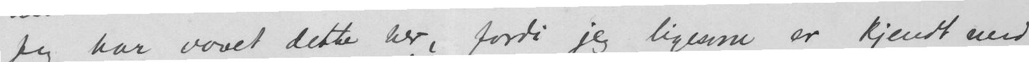Jeg har vovet dette her, fordi jeg ligesom er kjendt med
